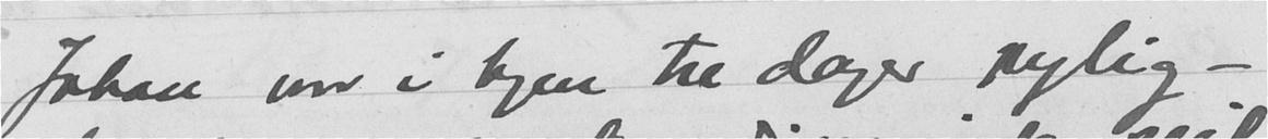Johan var i byen tre dager nylig -
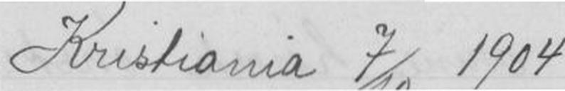Kristiania 7/10 1904
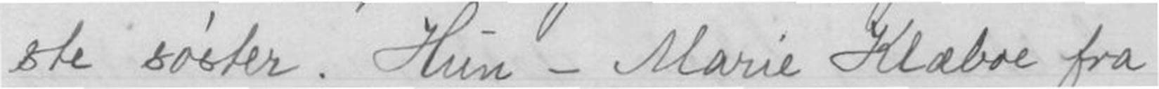ste søster. Hun - Marie Klæboe fra
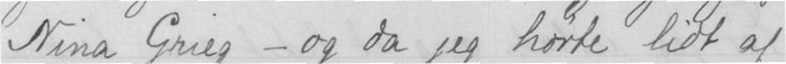Nina Grieg - og da jeg hørte lidt af
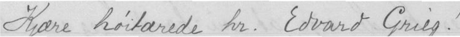Kjære høitærede hr. Edvard Grieg !
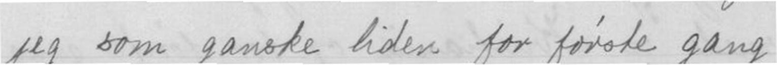jeg som ganske liden for første gang
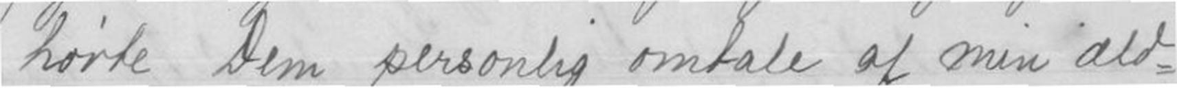hørte Dem personlig omtale af min Æld-
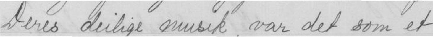Deres deilige musik, var det som et
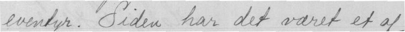eventyr. Siden har det været et af
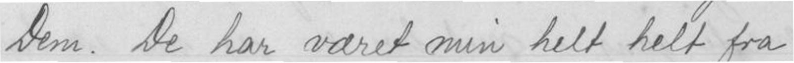Dem. De har været min helt helt fra
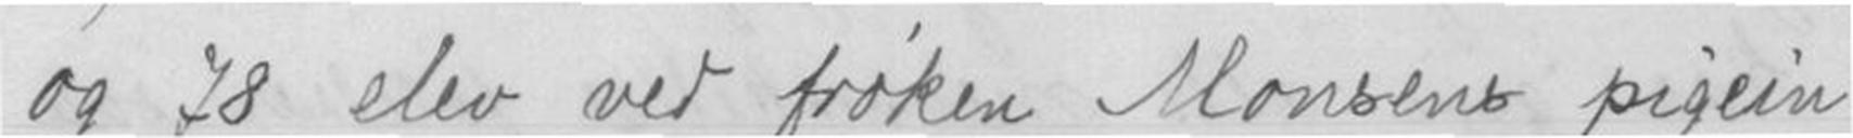og 78 elev ved frøken Monsens pigein-
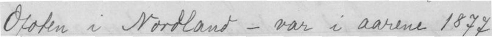Ofoten i Nordland - var i aarene 1877
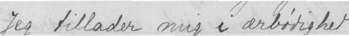Jeg tillader mig i ærbødighed
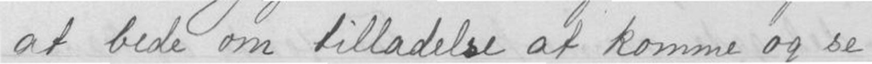at bede om tilladelse at komme og se
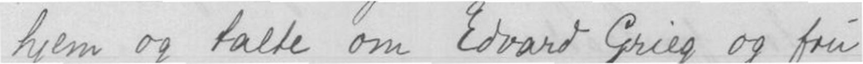hjem og talte om Edvard Grieg og fru
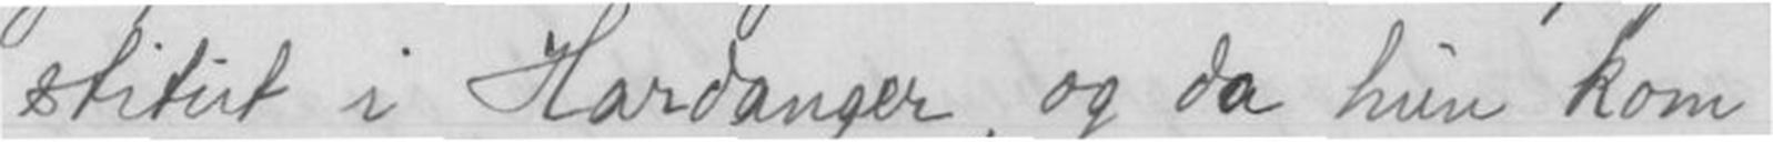stitut i Hardanger, og da hun kom
